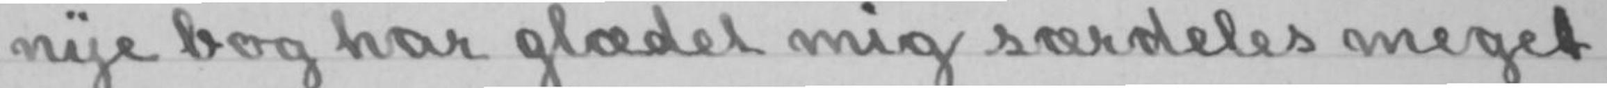nye bog har glædet mig særdeles meget
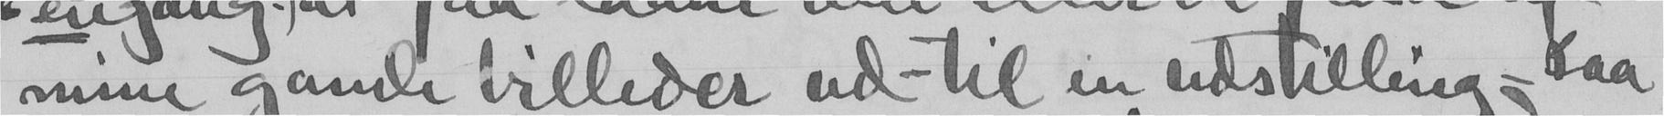mine gamle billeder ud til en udstilling,- saa
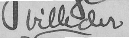billeder
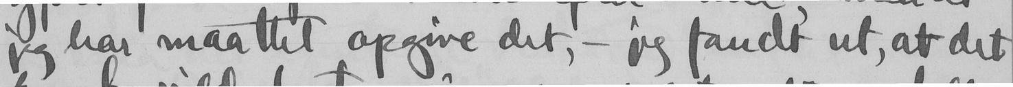jeg har maattet opgive det, - jeg fandt ut, at det
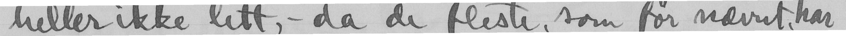heller ikke lett, - da de fleste, som før nævnt, har
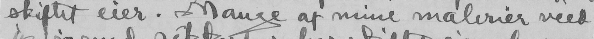skiftet eier. Mange af mine malerier veed
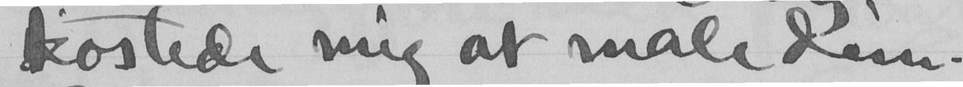kostede mig at male dem.
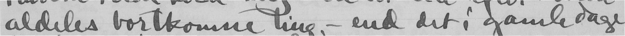aldeles bortkomne ting, - end det i gamle dage
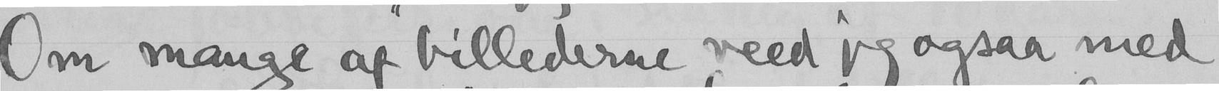Om mange af billederne veed jeg ogsaa med
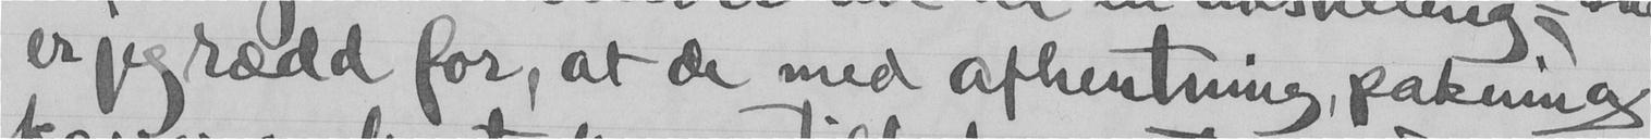er jeg rædd for, at de med afhentning, pakning,
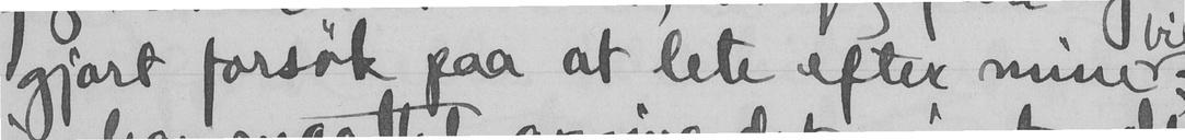gjort forsøk paa at lete efter mine.
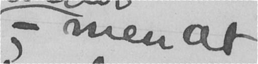-men at
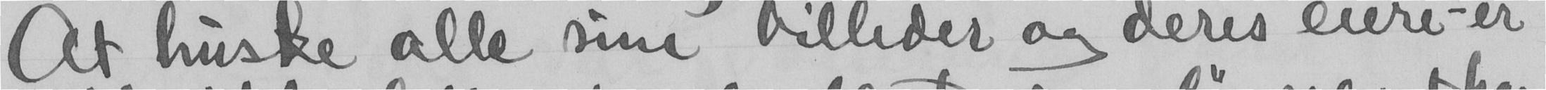At huske alle sine billeder og deres eiere - er
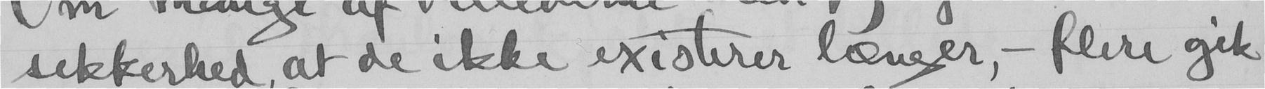sikkerhed, at de ikke existerer længer, - flere gik
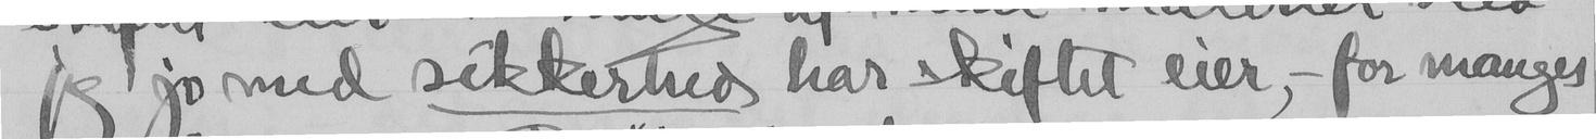jeg jo med sikkerhed har skiftet eier, - for manges
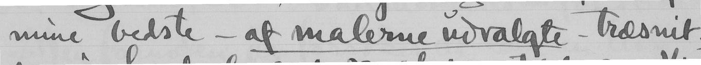mine bedste - af malerne udvalgte - træsni,t
